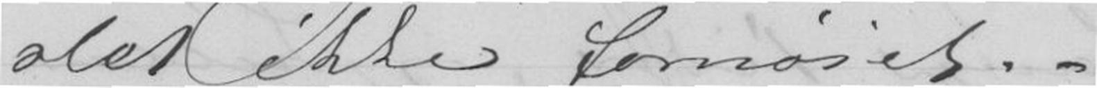slet ikke fornøiet. -
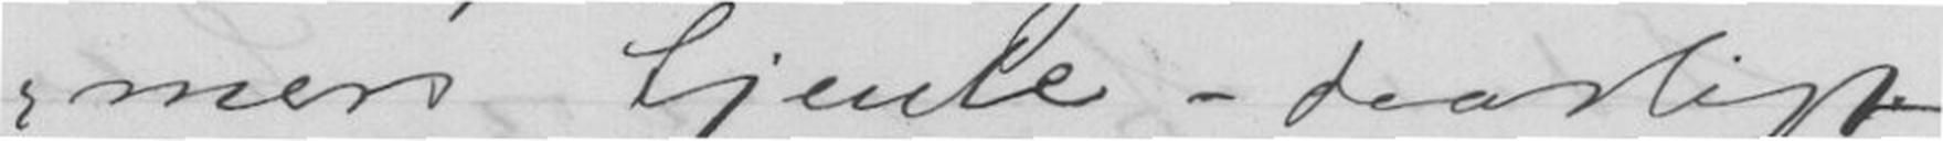mers tjente - daarligt
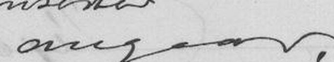angaar,
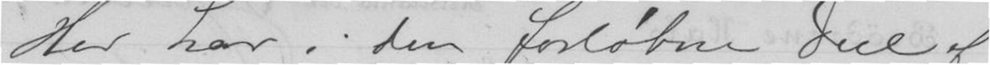Her har i den forløbne Deel af
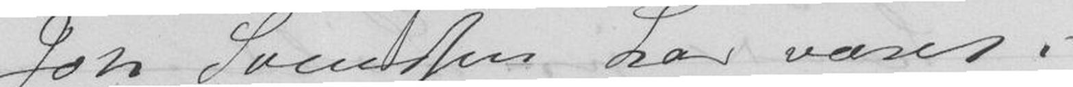Joh Svendsen har varet i
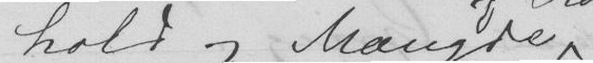hold og Mængde
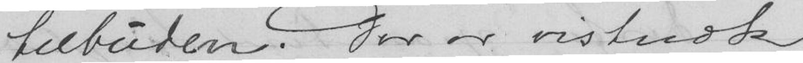tilbuden. Der er vistnok
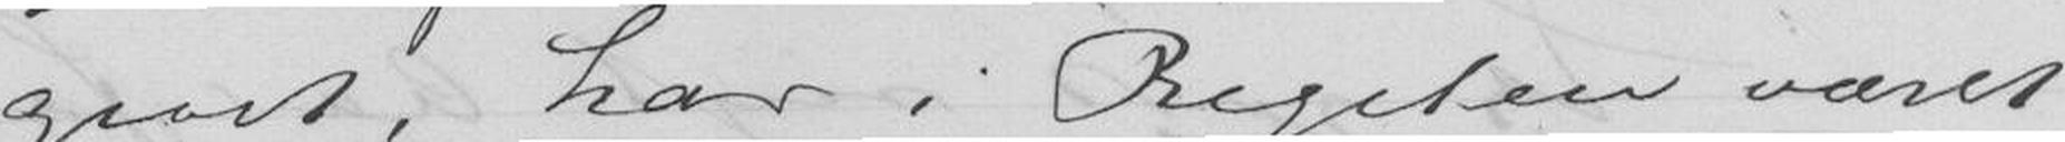givet, har i Regelen varet
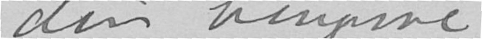din hengivne
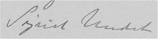Sigrid Undset
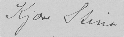Kjære Stina
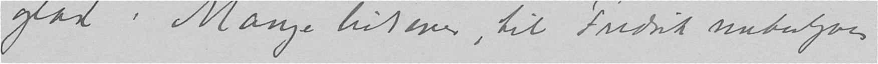glad? Mange hilsener, til Fredrik naturligvis
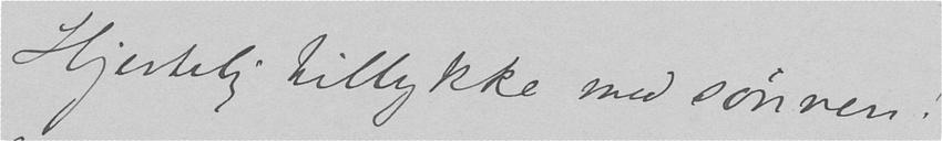Hjertelig tillykke med sønnen!
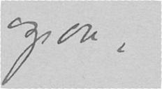ogsaa.
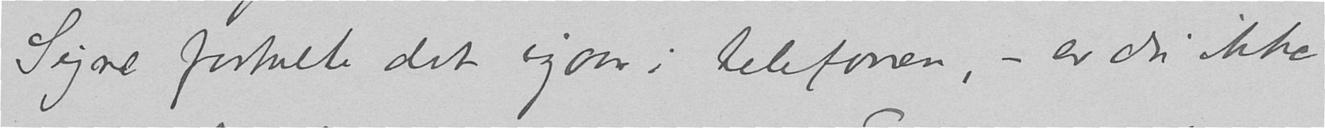Signe fortalte det igaar i telefonen - er du ikke
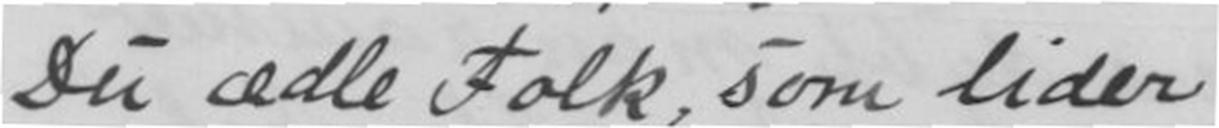Du ædle Folk, som lider
